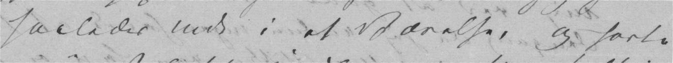saaledes inde i et Værelse, og hørte
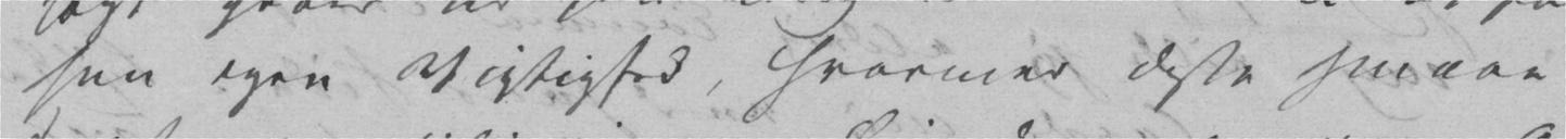sin egen Vigtighed, hvormed disse smaae
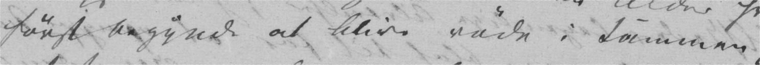først begynde at blive røde i Kammen.
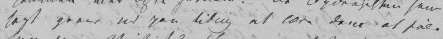sagt gaaer ud paa tidlig at lære dem at føle
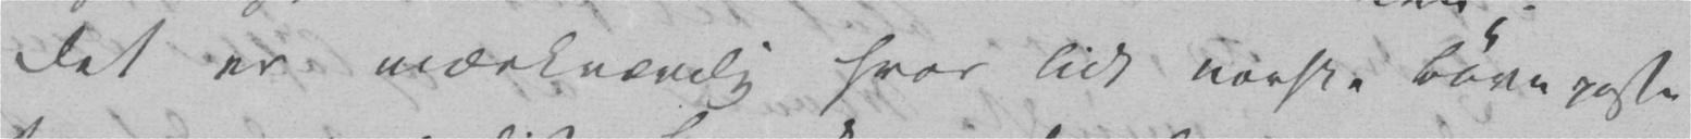Det er mærkværdig hvor lidt norske Børn passe
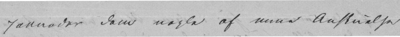paanøder Dem nogle af mine Anskuelser
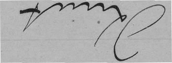Knut
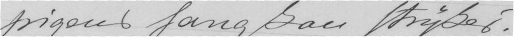av pigens sang kan stryges?
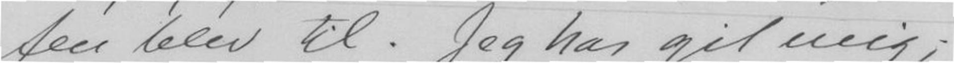fen blev til. Jeg har git mig;
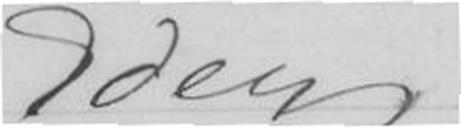Eders
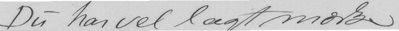Du har vel lagt mærke
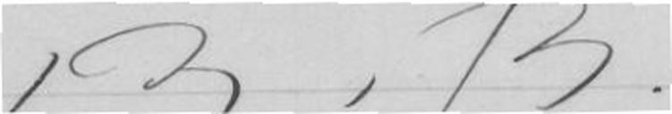B. B.
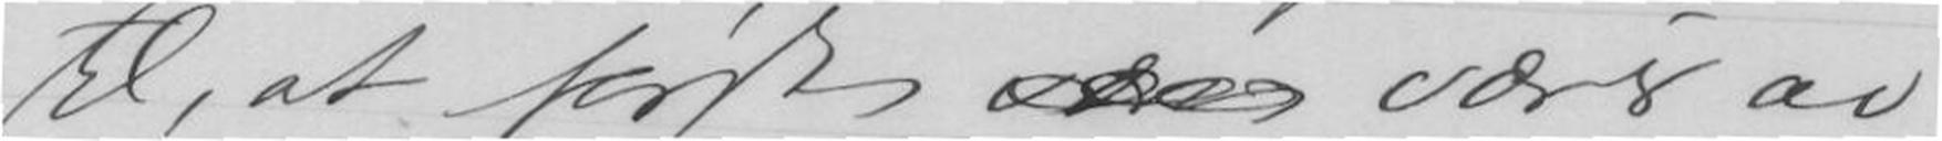til, at første være? værs
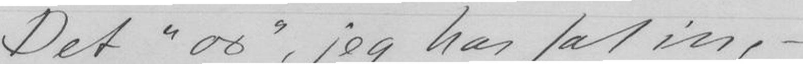Det "os", jeg har sat in, -
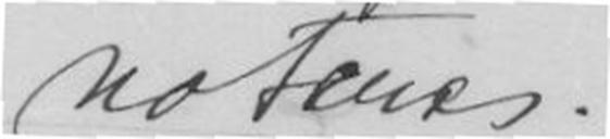noteres.
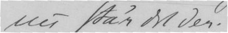nu står det der.
